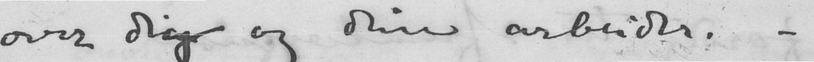over dig og dine arbeider. -
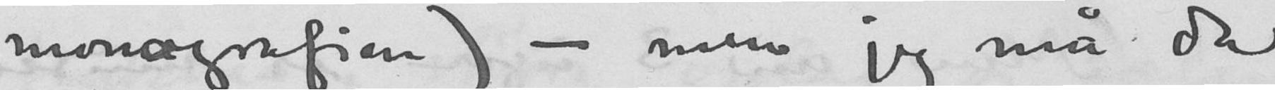monografien) - men jeg må da
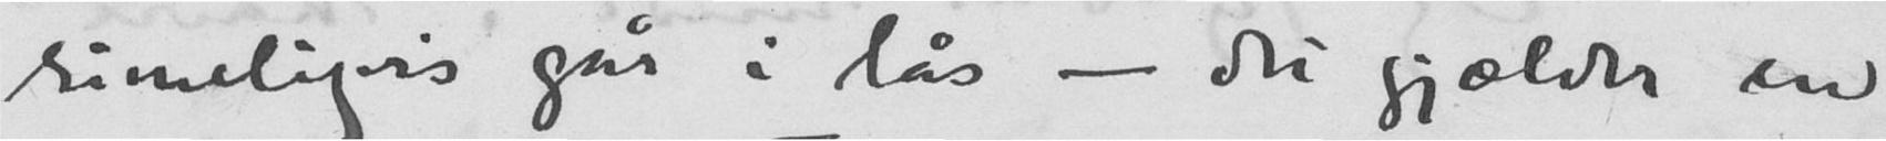rimeligvis går i lås - det gjælder en
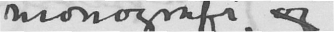monografi, og
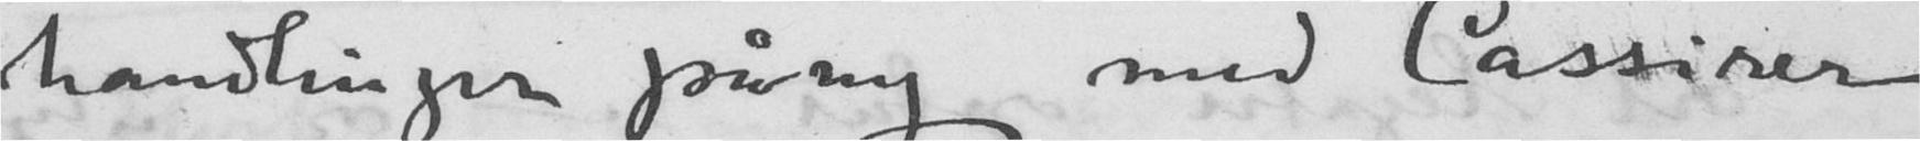handlinger påny med Cassirer
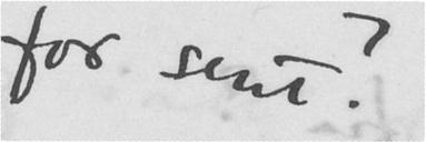for sent?
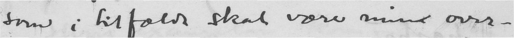som i tilfælde skal være min over-
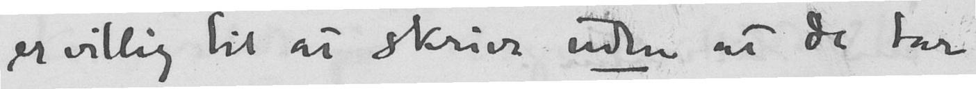er villig til at skrive uden at de tar
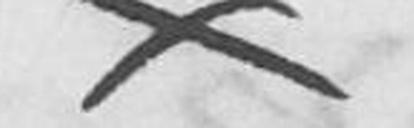X
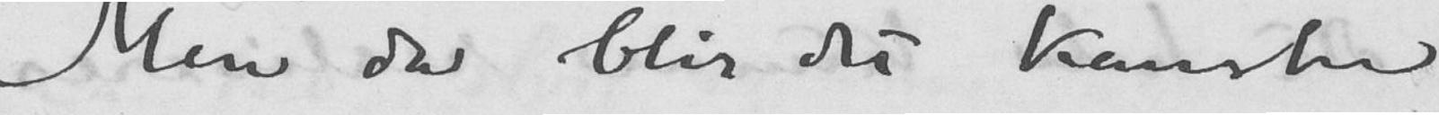Men da blir det kanske
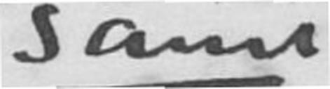samt
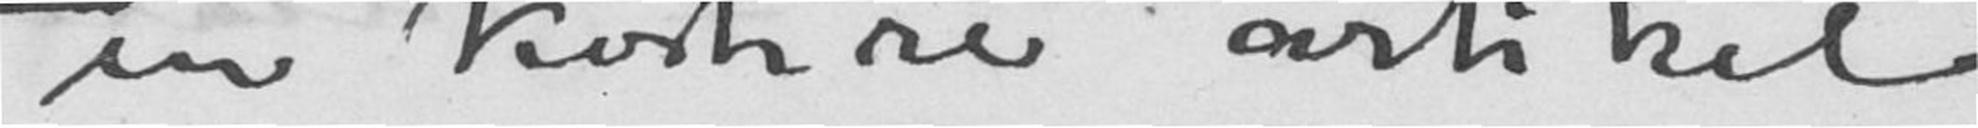en kortere artikel
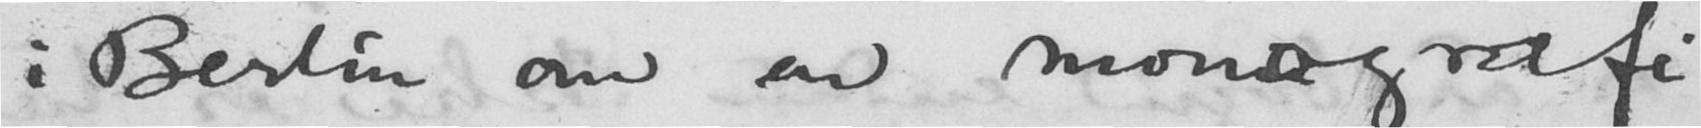i Berlin om en monografi
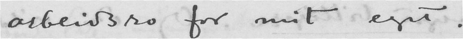arbeidsro for mit eget.
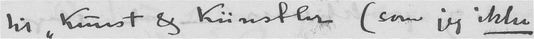til "Kunst og Künstler" (som jeg ikke
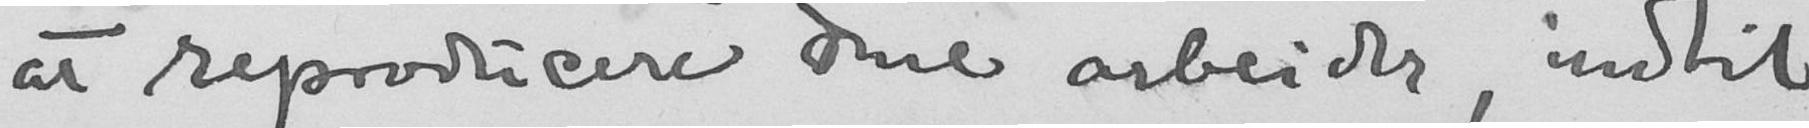at reproducere dine arbeider, indtil
